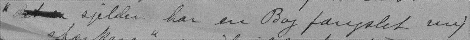"  sjelden har en Bog fængslet mig
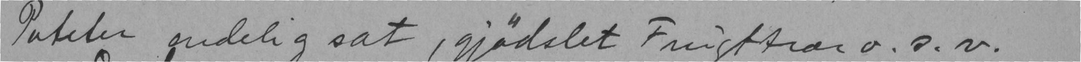Poteter endelig sat, gjødslet Frugttrær o. s.v.
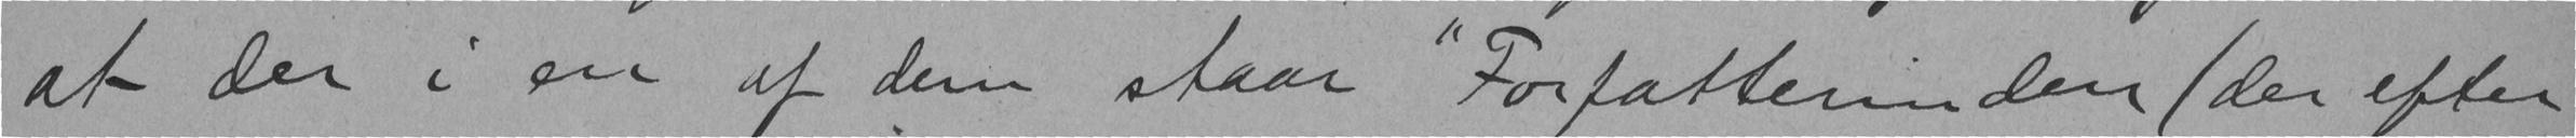at der i en af dem staar "Forfatterinden (der efter
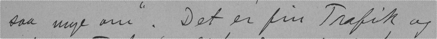saa mye om". Det er fin Trafik og
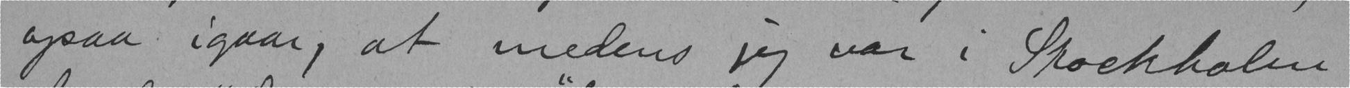ogsaa igaar, at medens jeg var i Stockholm
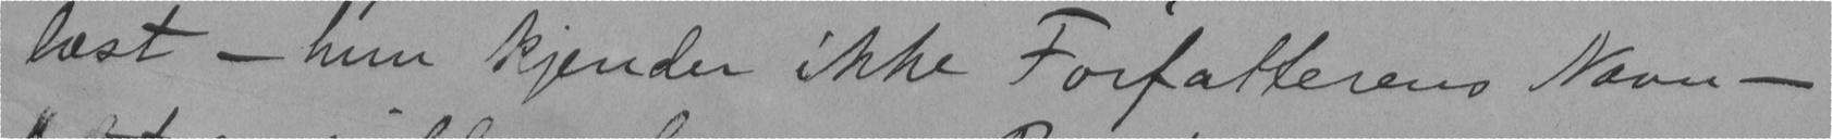læst - hun kjender ikke Forfatterens Navn -
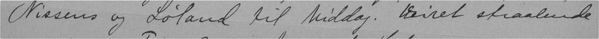Nissens og Løland til Middag. Veiret straalende
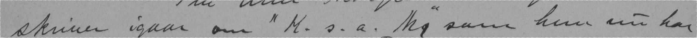skriver igaar om "K. s. a. M", som hun nu har
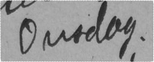Onsdag
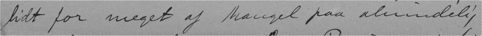lidt for meget af Mangel paa almindelig
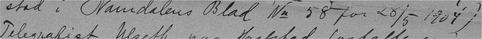stod i Namdalens Blad Nr 58 for 28/5 1904)
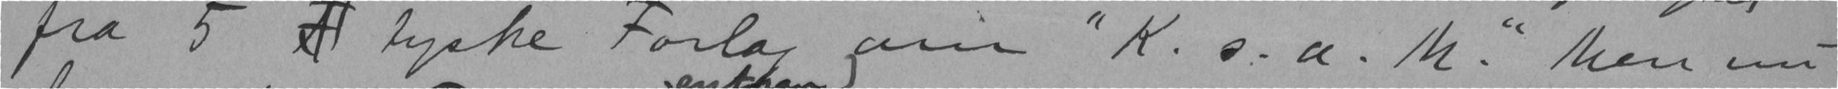fra 5  tyske Forlag om "K. s. a. M." Men nu
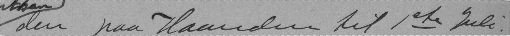den paa Haanden til 1ste Juli.
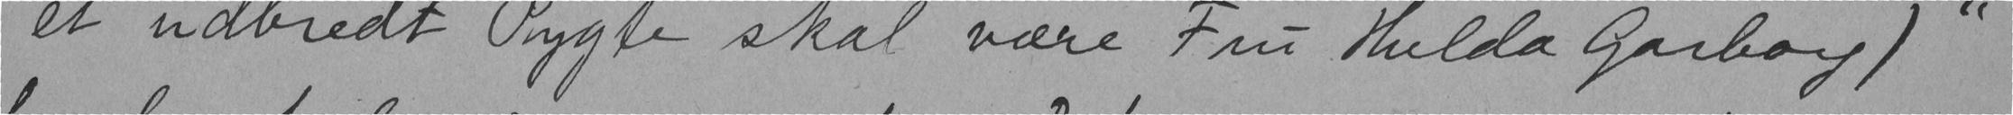et udbredt Rygte skal være Fru Hulda Garborg)"
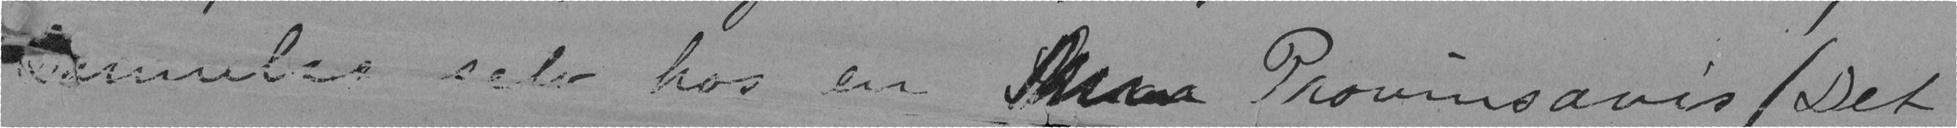semulae selv hos en  Provinsavis (Det
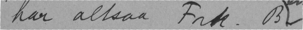har altsaa Frk. B
